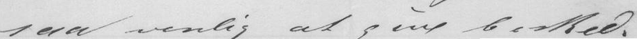saa venlig at give besked
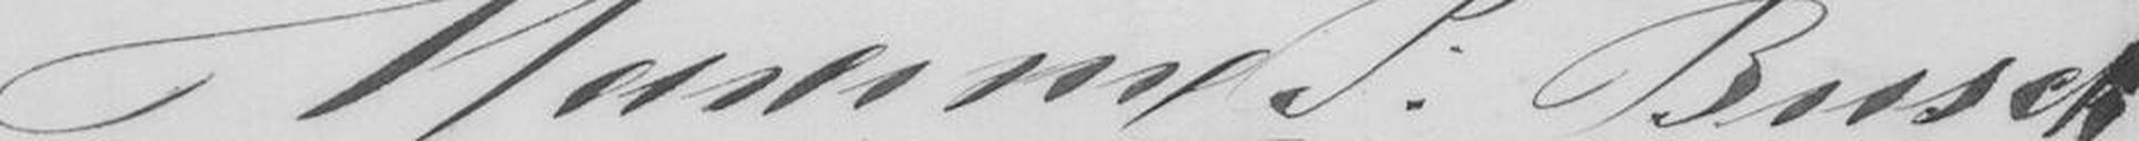Maxime P. Busch
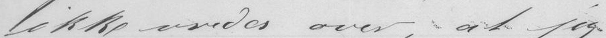ikke vredes over, at jeg
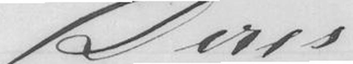Deres
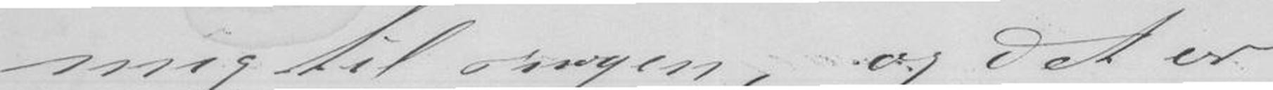mig til nogen, og det er
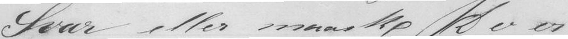Svar eller maaske De er
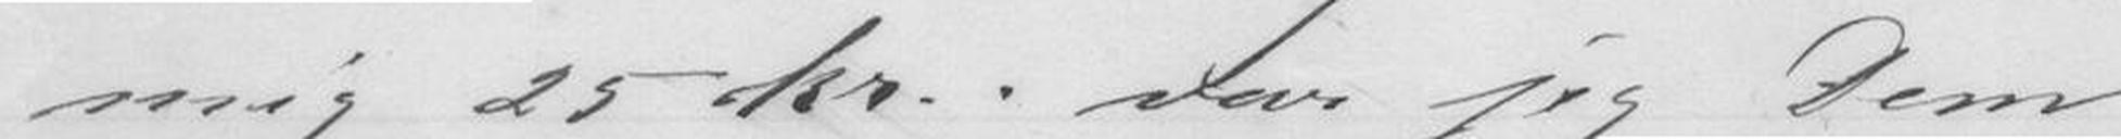mig 25 kr. var jeg Dem
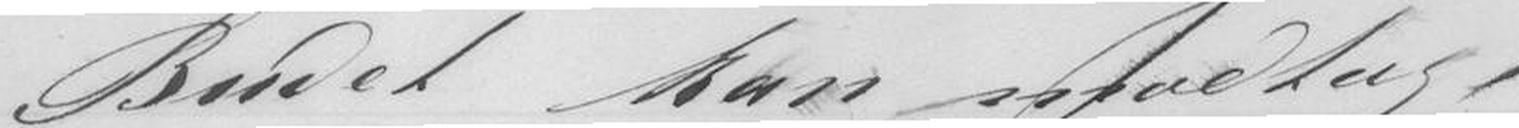Budet kan medtage
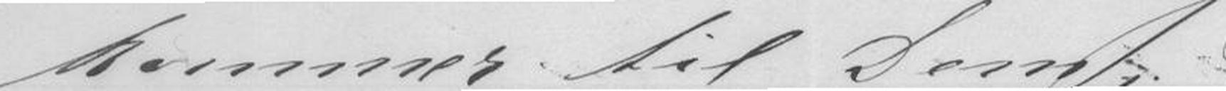kommer til Dem;
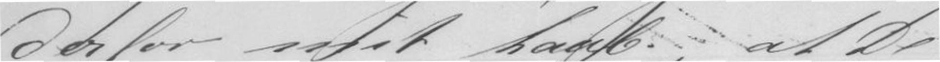derfor mit haab, at De
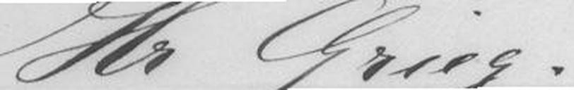Hr Grieg.
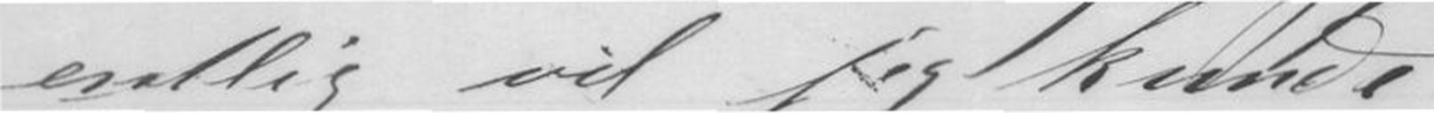entlig vil jeg kunde
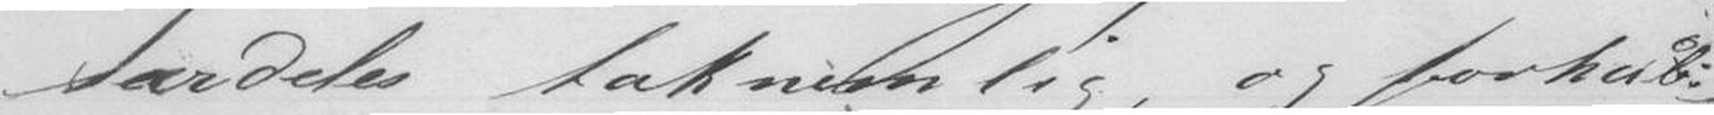særdeles taknemlig, og forhåb-
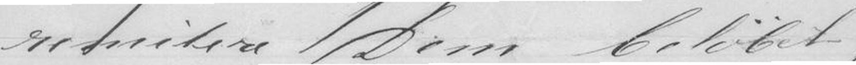remitere Dem beløbet,
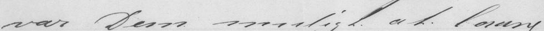var Dem muligt at laane
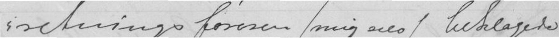retningsføreren /mig selv/ beklagede
